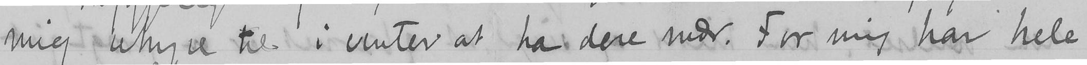mig uhyre til i vinter at ha dere nær. For mig har hele
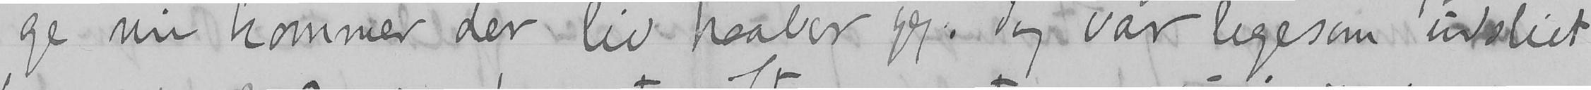ge nu kommer der liv haaber jeg. Jeg var ligesom udslidt
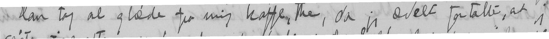Han tog al glæde fra mig kaffe, the, da jeg ædelt fortalte, at jeg
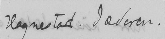Hognestad. Jæderen.
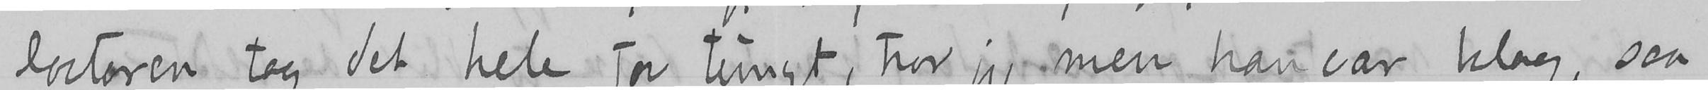Doctoren tog det hele for tungt, tror jeg, men han var klog, saa
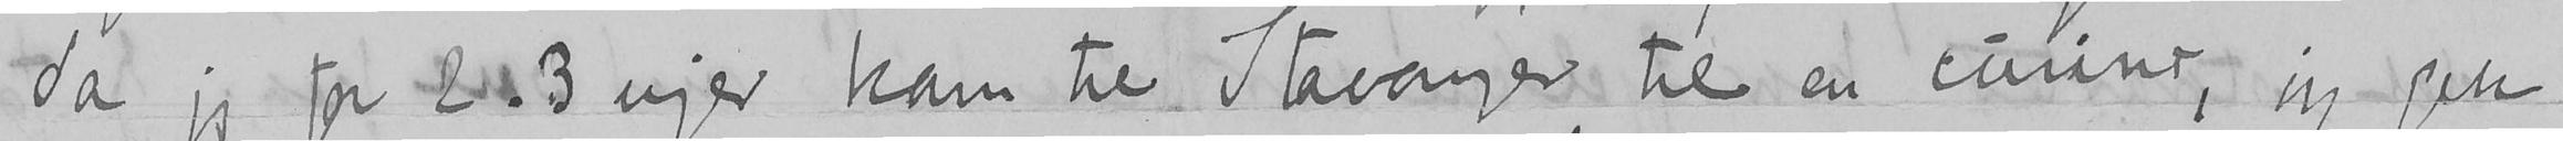da jeg for 2-3 uger kom til Stavanger til en cuunr, jeg gik
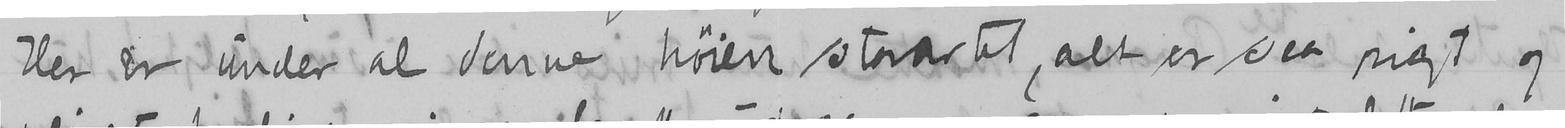Her er under al denne høien storartet, alt er saa rigt og
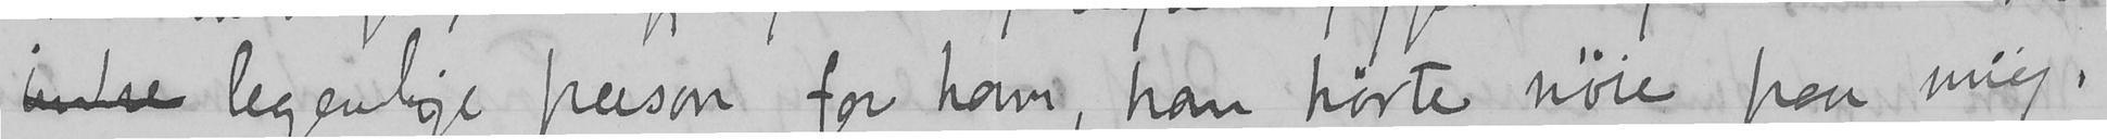ludse legemlige person for ham, han hørte nøie paa mig,
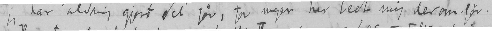jeg har aldrig gjort det før, for ingen har bedt mig derom før.
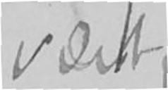været
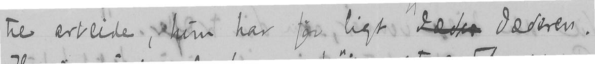til arbeide, hun har før ligt Jæder Jæderen.
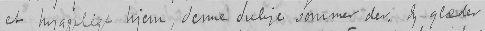et hyggeligt hjem, denne deilige sommer der. Jeg glæder
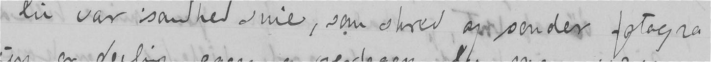Du var i sandhed snil, som skrev og sender fotogra-
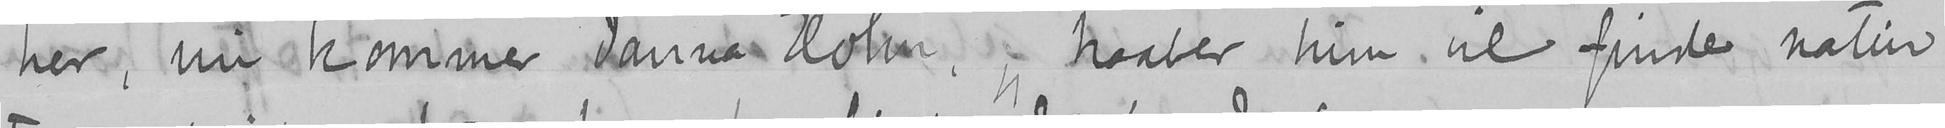her, nu kommer Janna Holm, jeg haaber hun vil finde natur
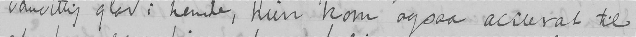vanvittig glad i hende, hun kom ogsaa accurat til
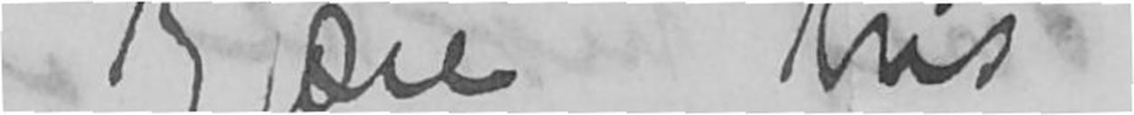Kjære Kris
